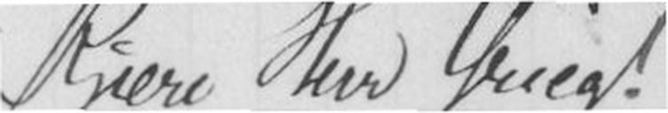Kjære Herr Grieg!
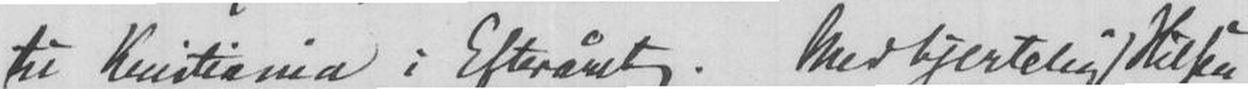til Kristiania i Efteråret. Med hjertelig Hilsen
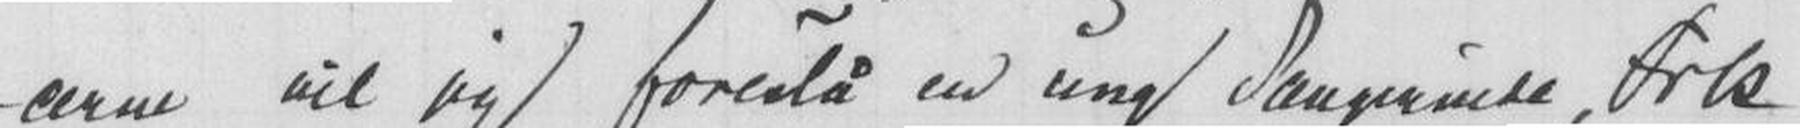cerne vil jeg foreslå en ung Sangerinde, Frk
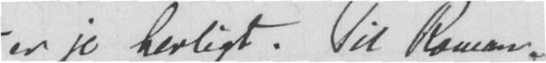er jo herligt. Til Roman-
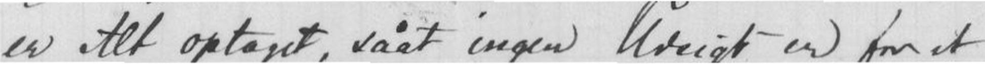er det optaget, såat ingen Udsigt er for at
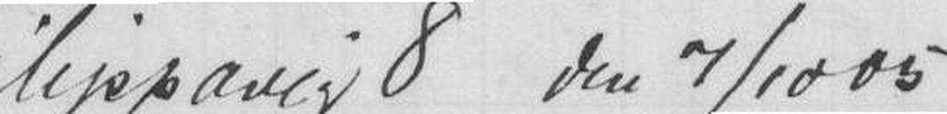lippavig 8 den 7/10 -05
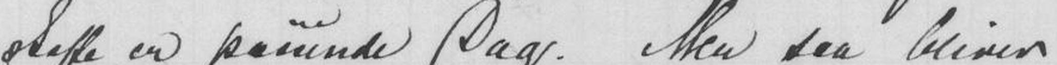skaffe er pan Dag.Men saa bliver
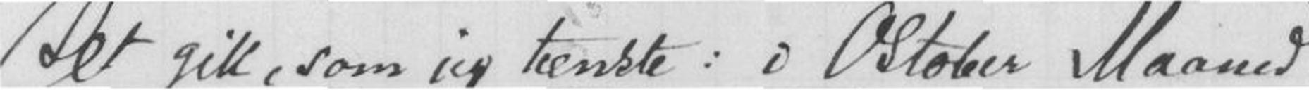Det gikk, som jeg tenkte: i Oktober Maaned
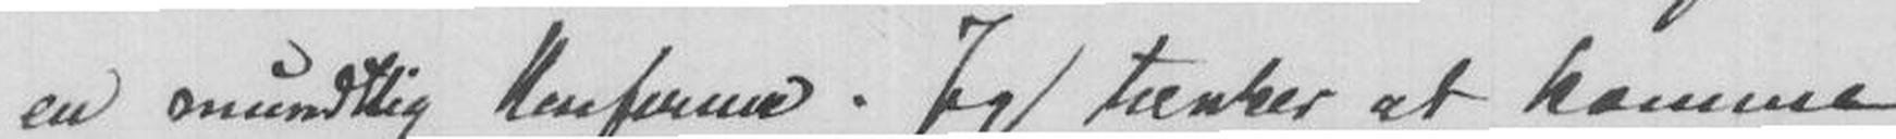en mundtlig Konferanse. Jeg tænker at komme
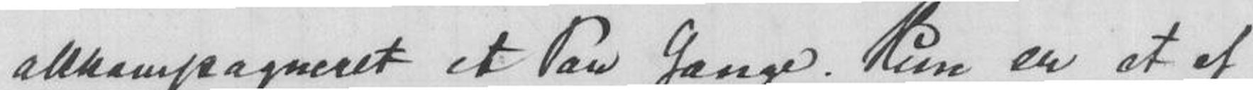akkompagneret et Par Gange. Hun er et af
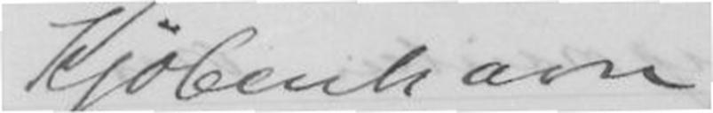Kjøbenhavn
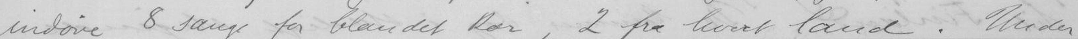indøve 8 sange for blandet kor, 2 fra hvert land. Under
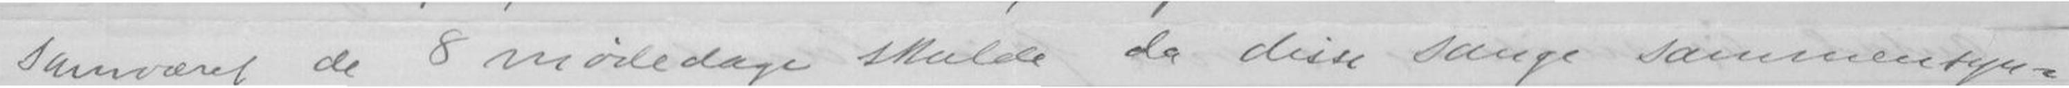samværet de 8 mødedage skulde da disse sange sammensyn-
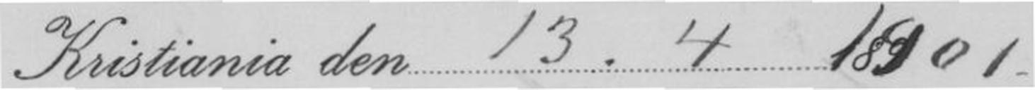Kristiania den 13.4 1901
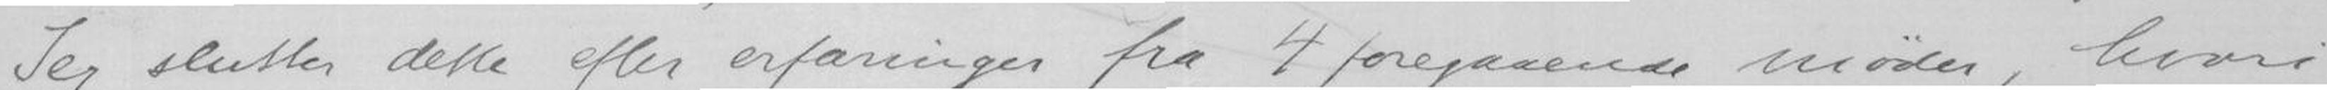Jeg slutter dette efter erfaringer fra 4 foregaaende møder, hvori
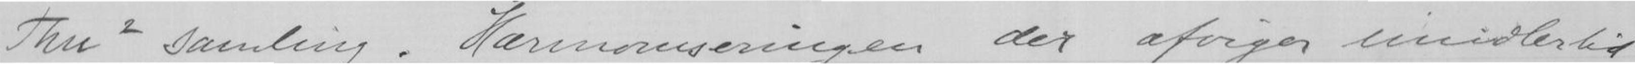Thu samling. Harmonseringen der afviger imidlertid
